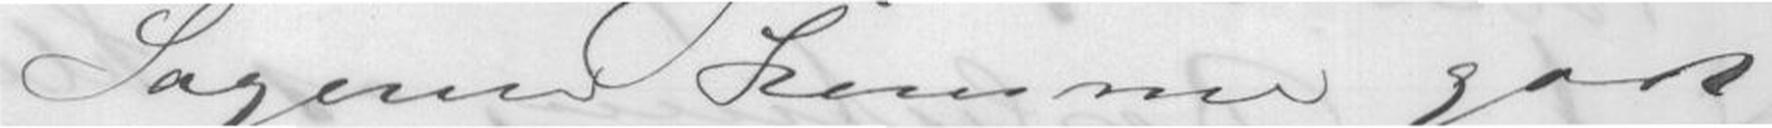Sagerne kommer godt
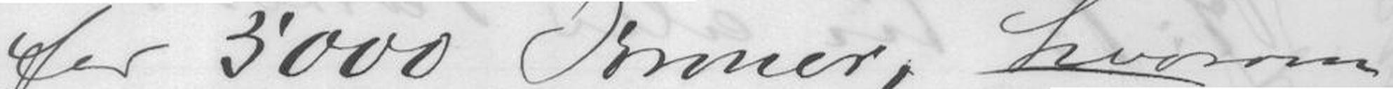for 5000 Kroner, hvorom
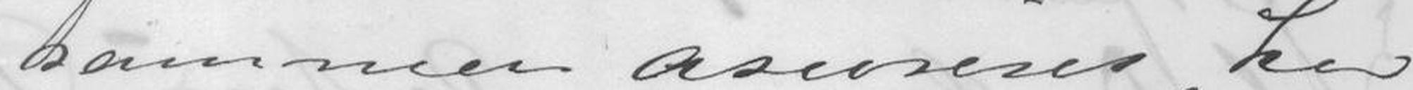sammen asureres her
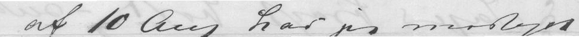af 10 Aug har jeg modtaget
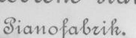Pianofabrik.
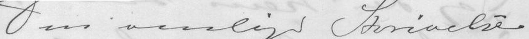Din venlige Skrivelse
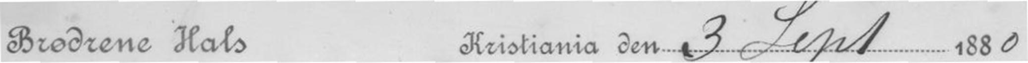Brødrene Hals Kristiania den 3 Sept 1880
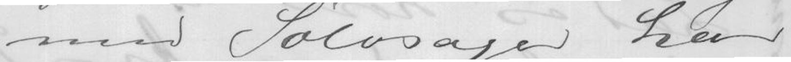med Sølvsager har
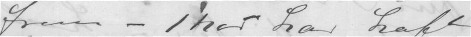frem - Thor har haft
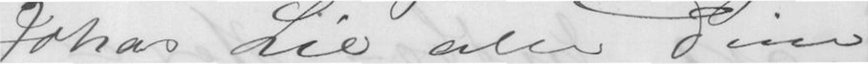Jonas Lie alle Dine
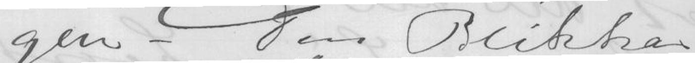gen - Den Blikka
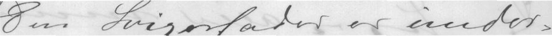Din Svigerfader er under-
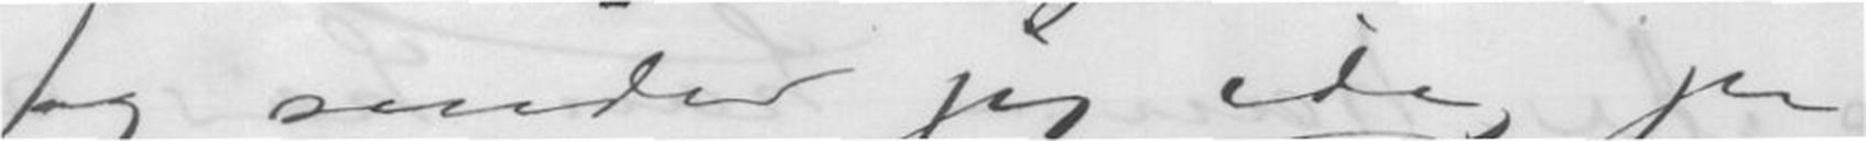og sender jeg idag pr.
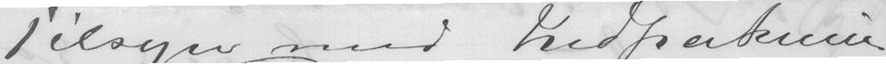Tilsyn med Indpaknin
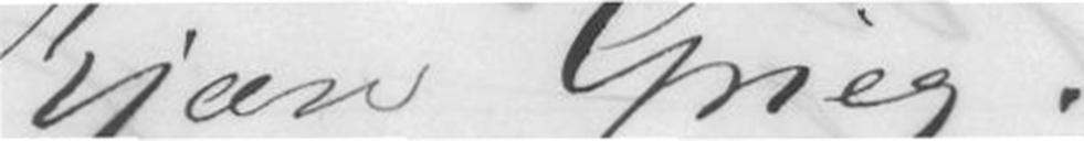Kjære Grieg!
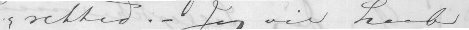retted. - Jeg vil haabe
